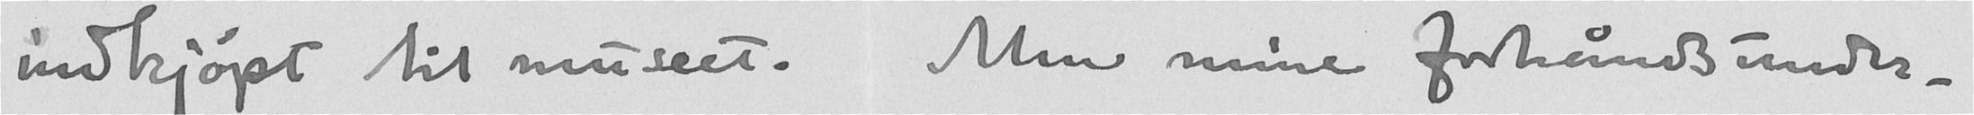indkjøpt til museet. Men mine forhåndsunder-
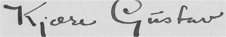Kjære Gustav
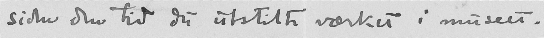siden den tid du utstilte værket i museet.
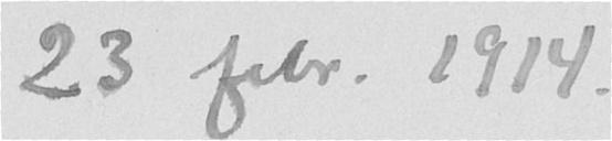23 febr. 1914.
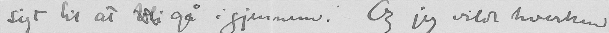sigt til at bli gå i gjennem. Og jeg vilde hverken
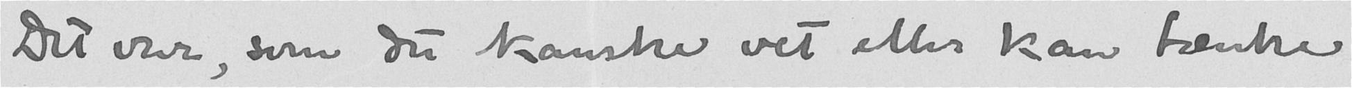Det var, som du kanske vet eller kan tænke
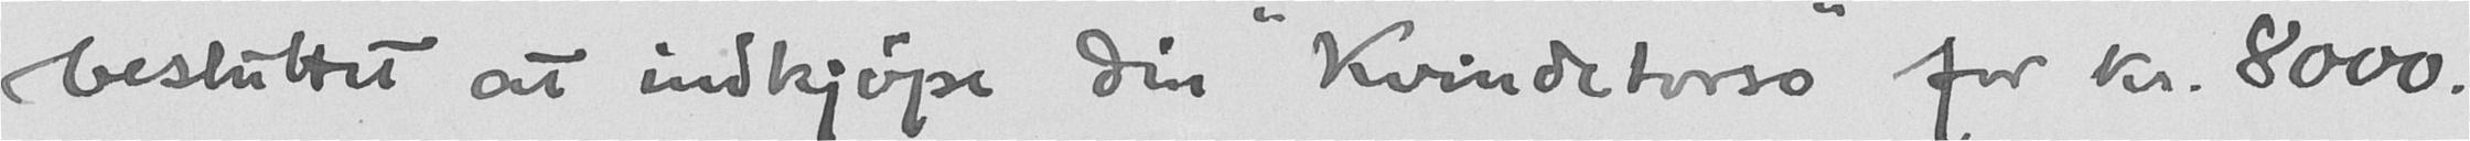besluttet at indkjøpe din "Kvindetorso" for kr. 8000.
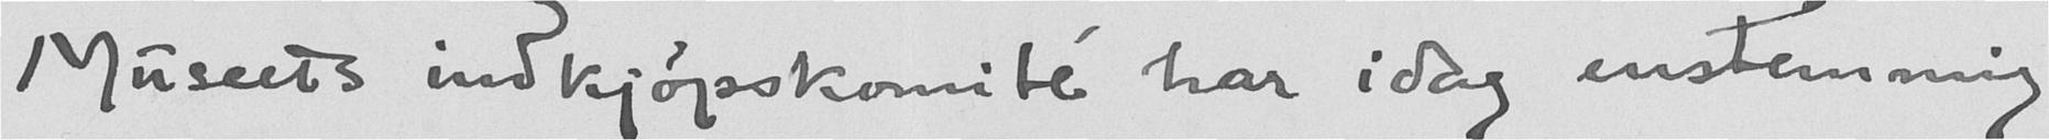Museets indkjøpskomité har idag enstemmig
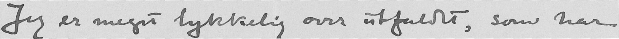Jeg er meget lykkelig over utfaldet, som har
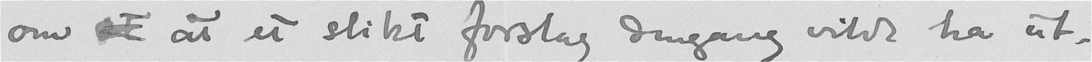om at at et slikt forslg dengang vilde ha ut-
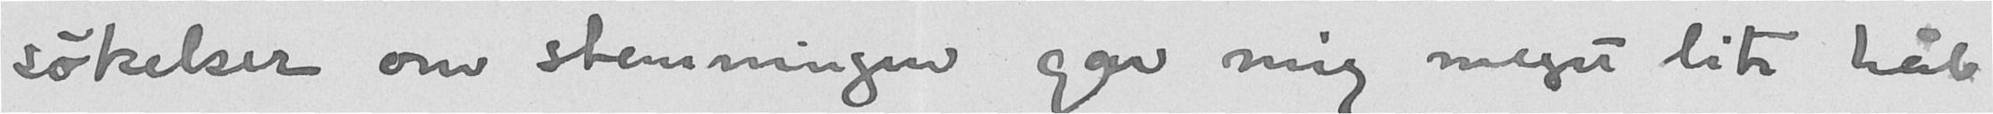søkelser om stemmingen gav mig meget lite håb
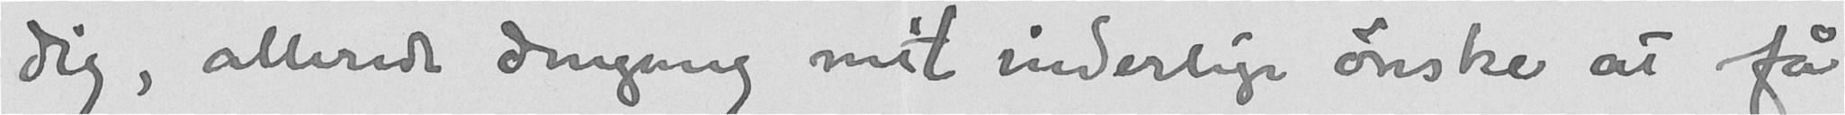dig, allerede dengang mit inderlige ønske at få
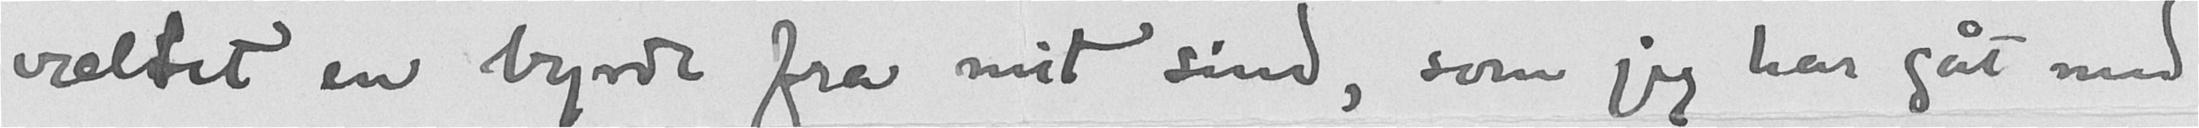væltet en byrde fra mit sind, som jeg har gåt med
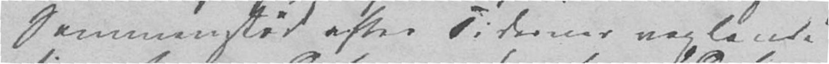Sammenstød efter Tidernes vexlende
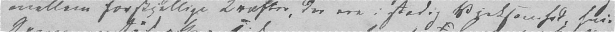mellem forskjellige Kræfter, der ere i stadig Virksomhed, hvis
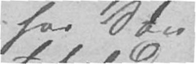for Søn-
